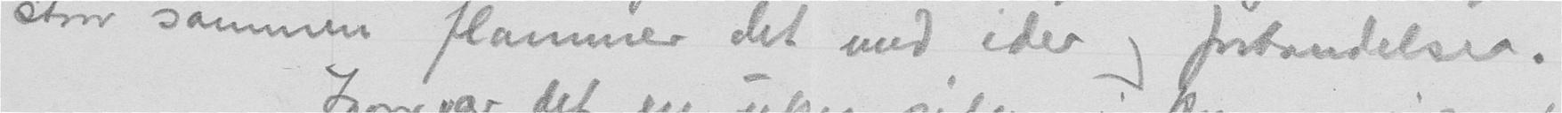staar sammen flammer det med ider og forbandelser.
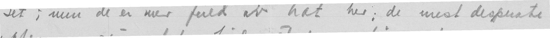set; men de er mer fuld av hat her; de mest desperate
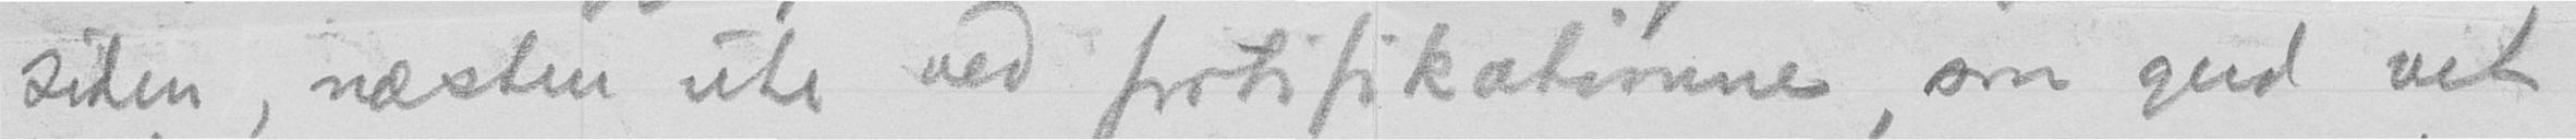siden, næsten ute ved fortifikationerne, saa gud vet
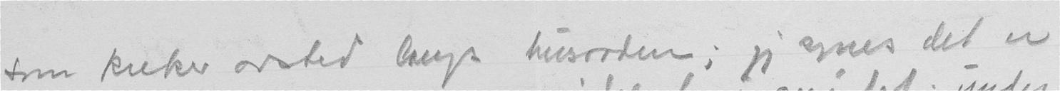som kreker avsted langs husraden; jeg synes det er
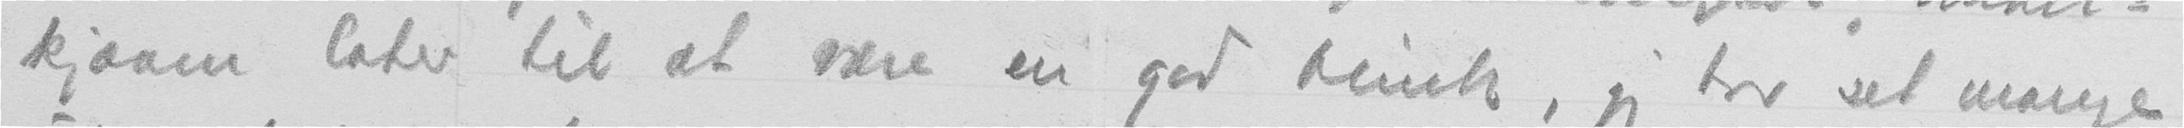kjæven later til at være en god blink, jeg har set mange
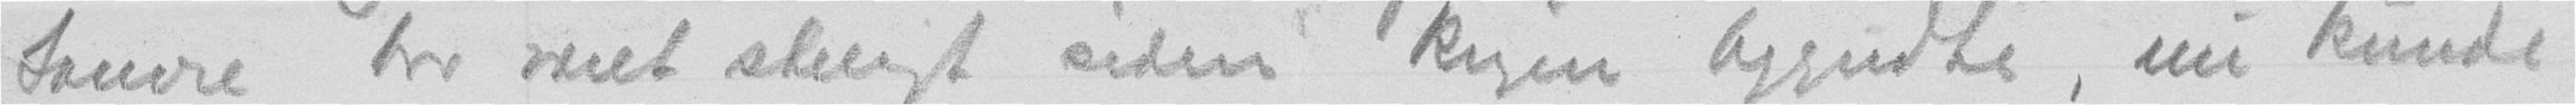Louvre har været stengt siden krigen begyndte, nu kunde
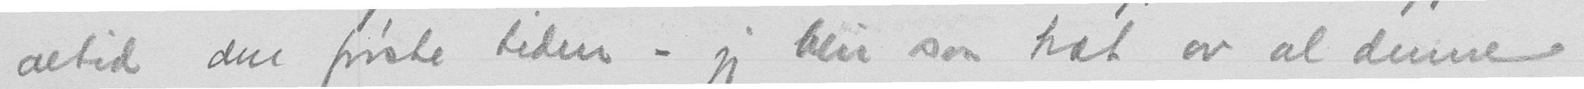altid den første tiden - jeg blir saa træt av al denne
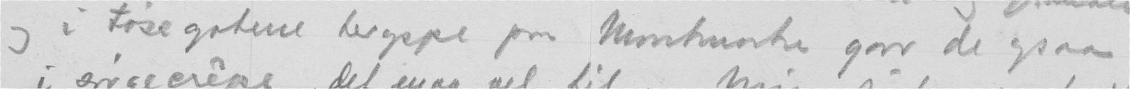og i tøsegatene heroppe paa Montmartre gaar de gsaa
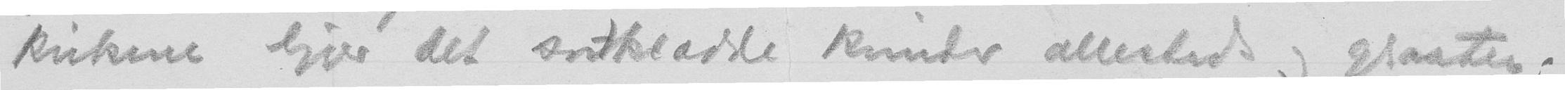kirkene ligger det sortklædde kvinder allesteds og graater
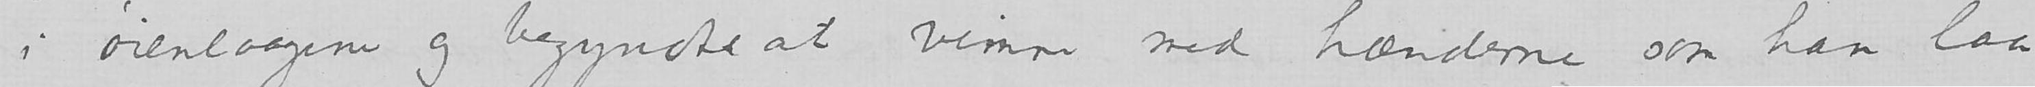i øienlaagene og begyndte at vimre med hænderne som han laa
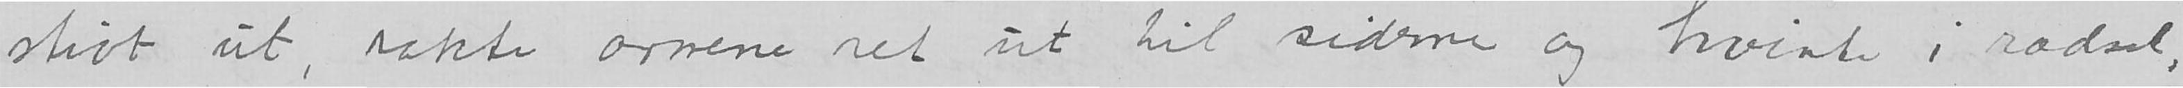stivt ut, rakte armene ned ut til siderne og hvinte i rædsel,
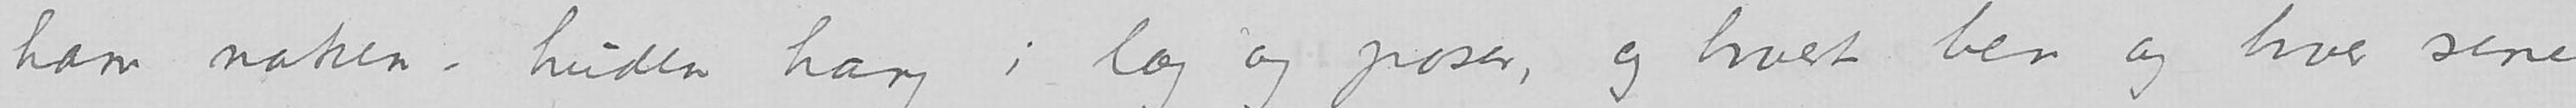ham naken – huden hang i læg og poser, og hvert ben og hver sene
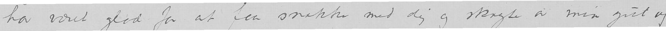har været glad for at faa snakke med dig og skryte av min gut og
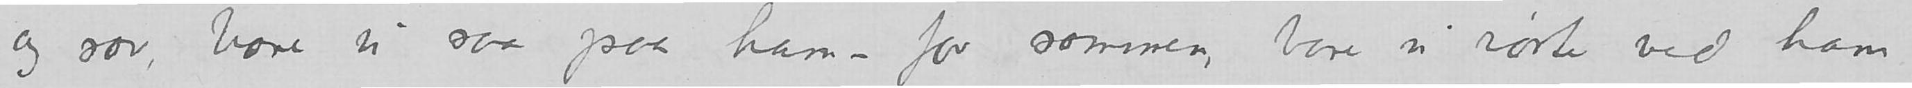og sov, bare vi saa paa ham – for sammen, bare vi rørte ved ham,
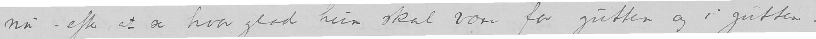nu – efter at sde hvor glad hun skal være for gutten og i gutten.
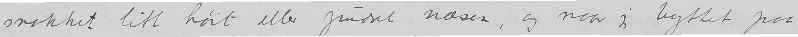snakket litt høit eller pudset næsen, og naar jeg byttet paa
